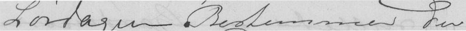Lørdagen. Bestemmer Du
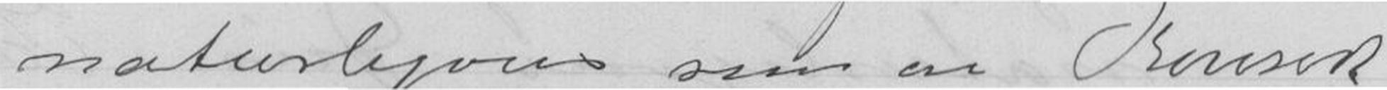naturligøres som en Konsert
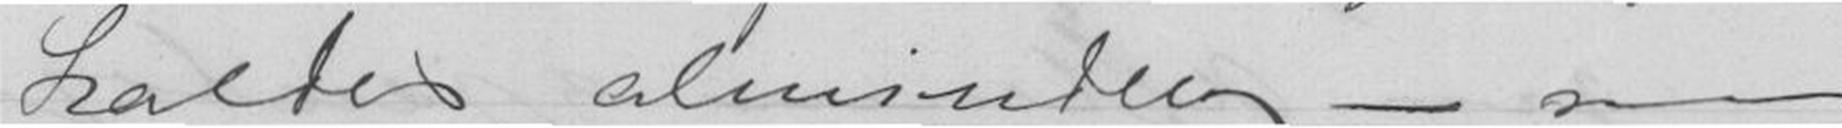kaldes almindelig - saa
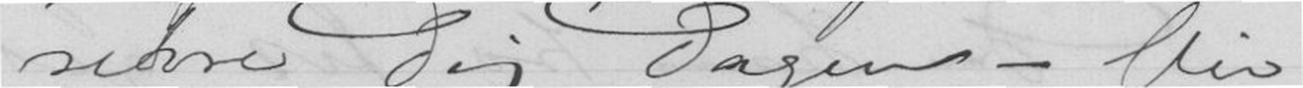sikre Dig Dagen - Vil
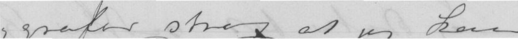grafer strax at jeg kan
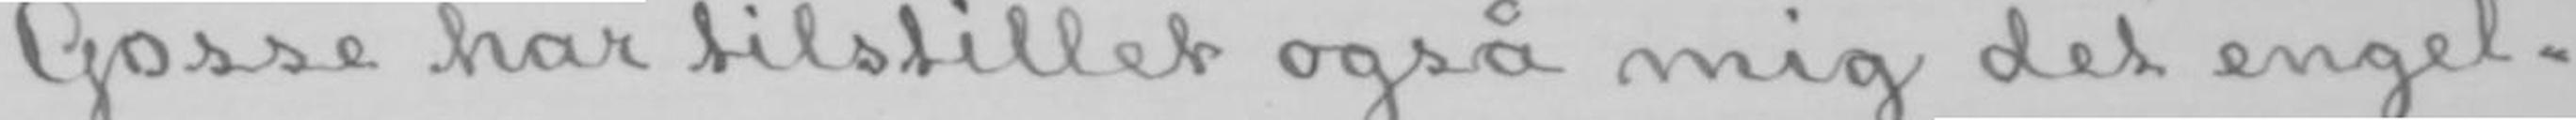Gosse har tilstillet også mig det engel-
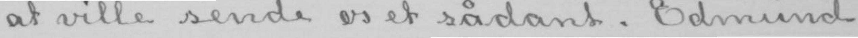at ville sende os et sådant. Edmund
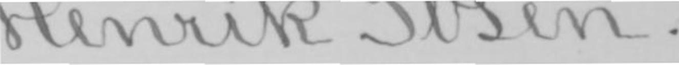Henrik Ibsen.
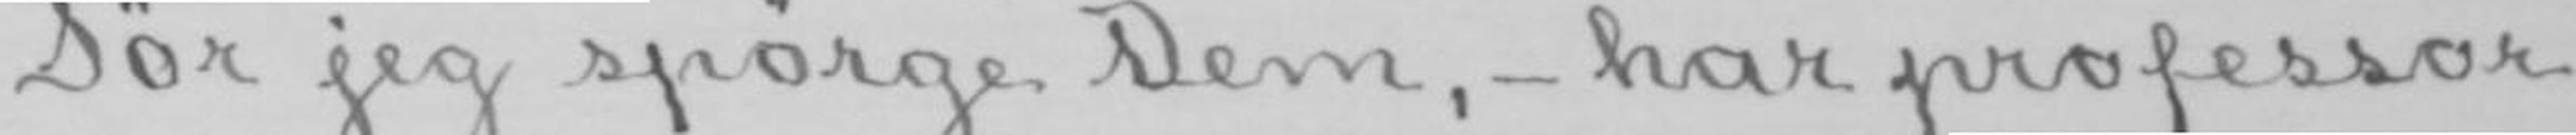Tør jeg spørge Dem,- har professor
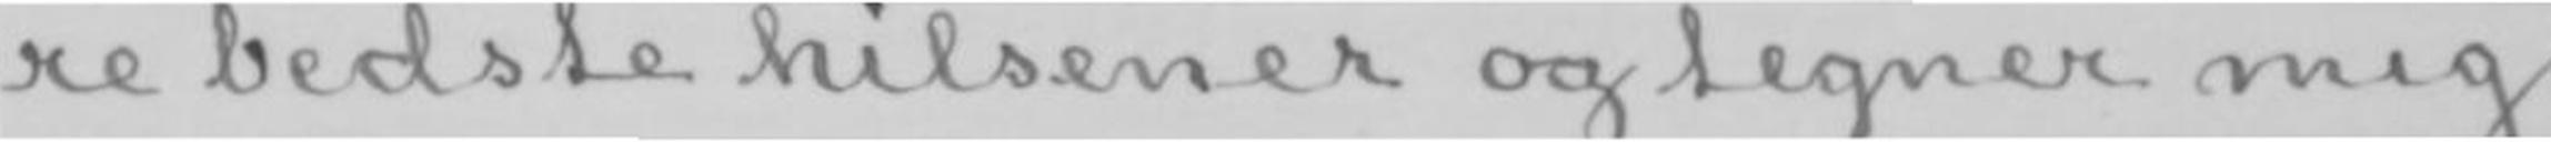re bedste hilsener og tegner mig
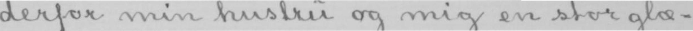derfor min hustru og mig en stor glæ-
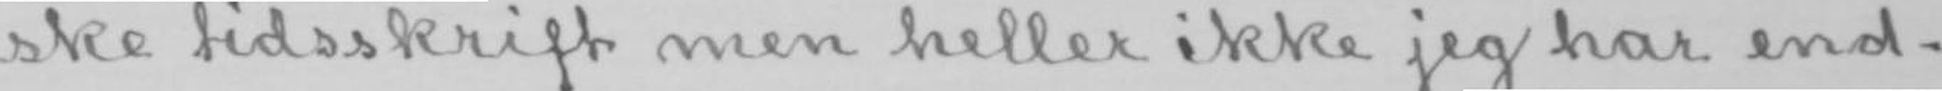ske tidsskrift men heller ikke jeg har end-
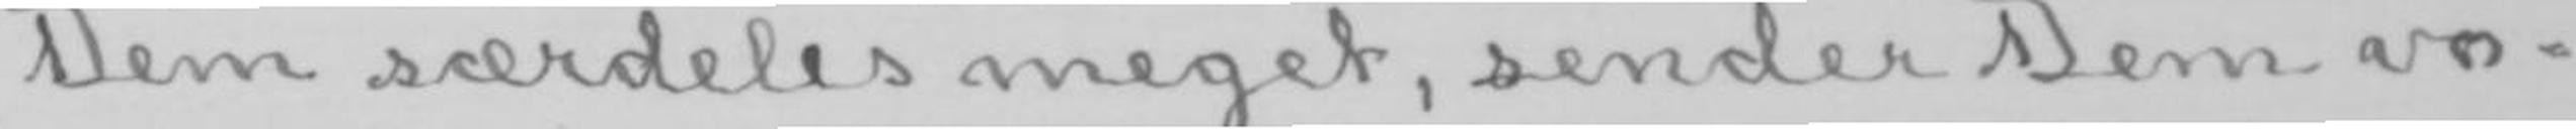Dem særdeles meget, sender Dem vo-
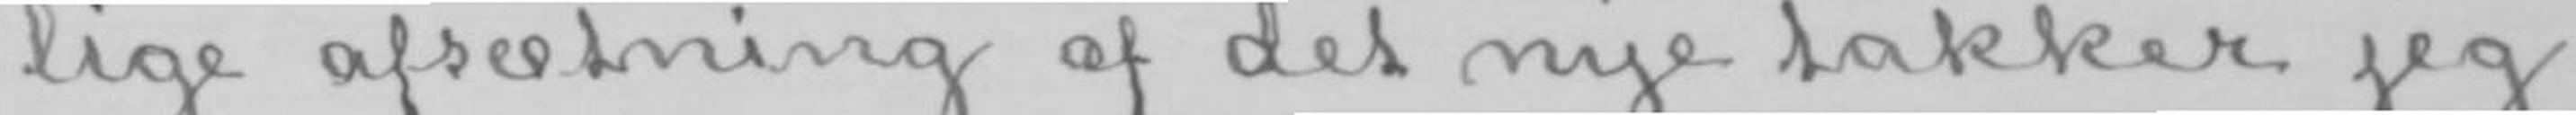lige afsætning af det nye takker jeg
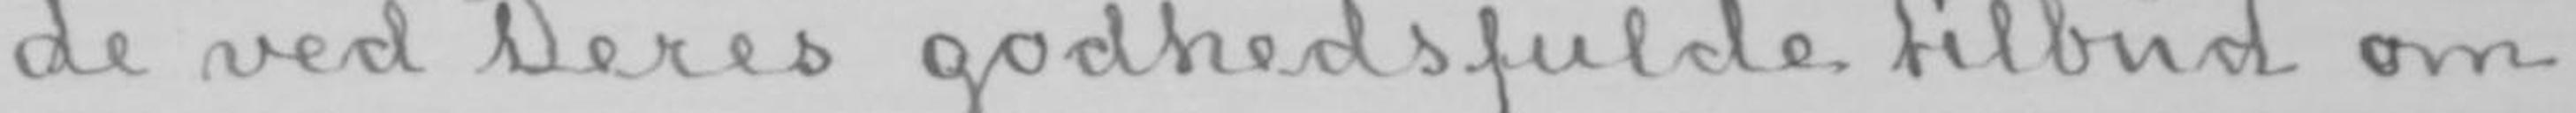de ved Deres godhedsfulde tilbud om
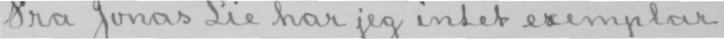Fra Jonas Lie har jeg intet exemplar
